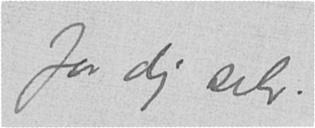for dig selv.
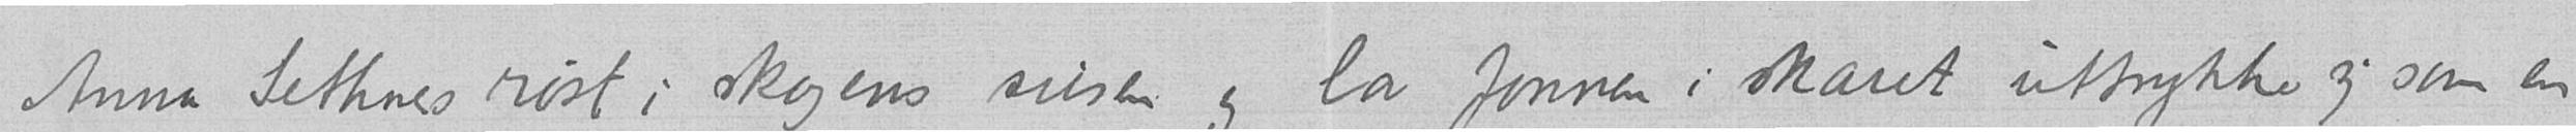Anna Sethnes røst i skogens susen og la fonnen i skaret uttrykke sig som en
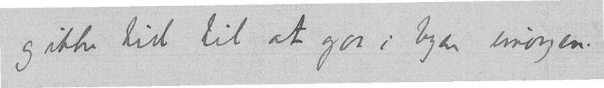og ikke tid til at gaa i byen imorgen.
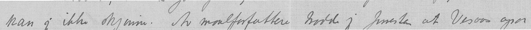kan jeg ikke skjønne. Av maalforfattere trodde jeg forresten at Vesaas ogsaa
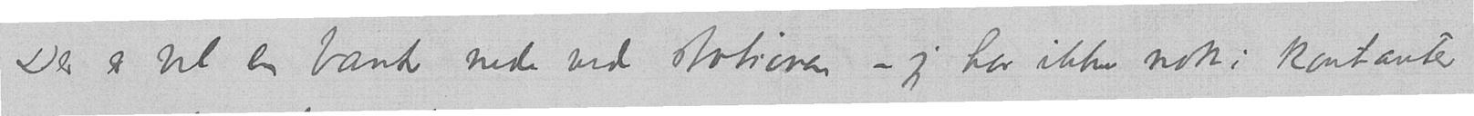Der er vel en bank nede ved stationen – jeg har ikke nok i kontanter
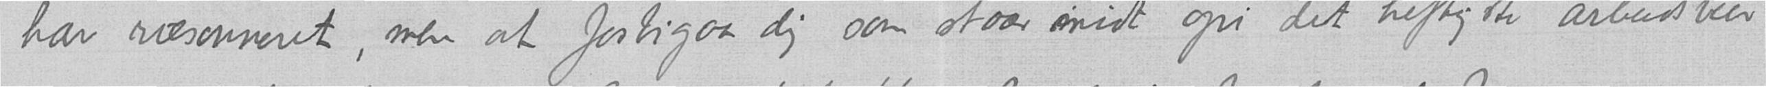har ræsonneret, men at forbigaa dig som staar midt opi det heftigste arbeidsveir
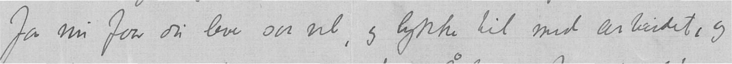Ja nu faar du leve saa vel, og lykke til med arbeidet, og
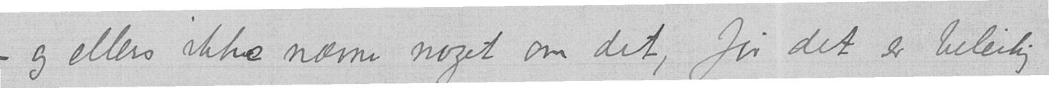– og ellers ikke nævne noget om det, før det er beleilig
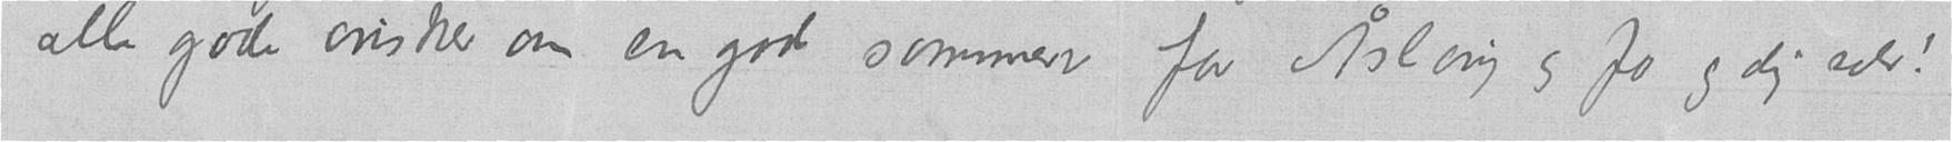alle gode ønsker om en god sommer for Åslaug og Jo og dig selv!
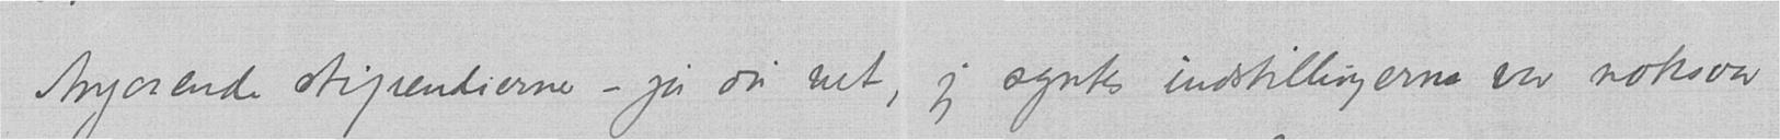Angaaende stipendierne – ja du vet, jeg syntes indstillingerne var noksaa
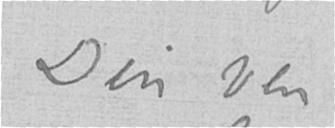Din Ven
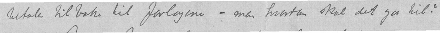betales tilbake til forlagene – men hvordan skal det gaa til?
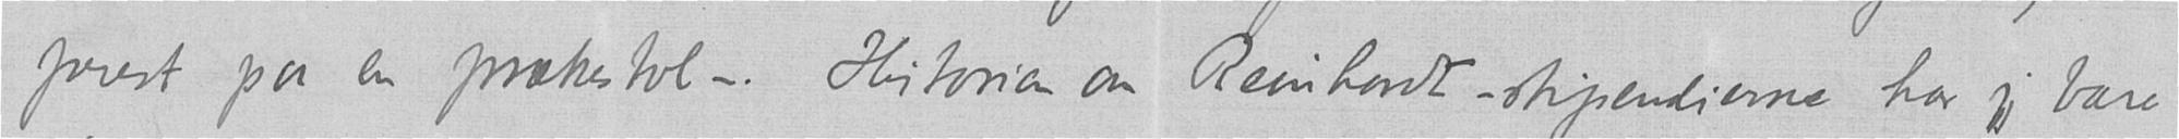prest paa en prækestol –. Historien om Reinhardt-stipendierne har jeg bare
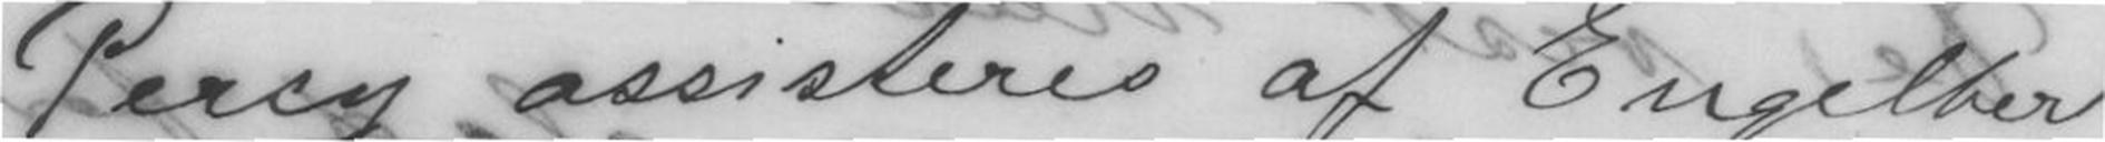Percy assisteres af Engelbert
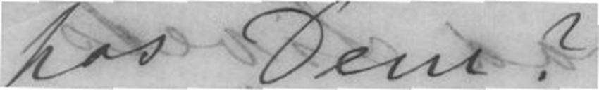hos Dem?
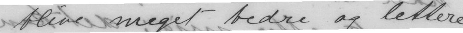blive meget bedre og lettere
